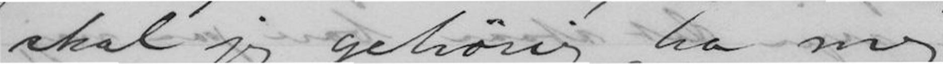skal jeg gehørig ha mig
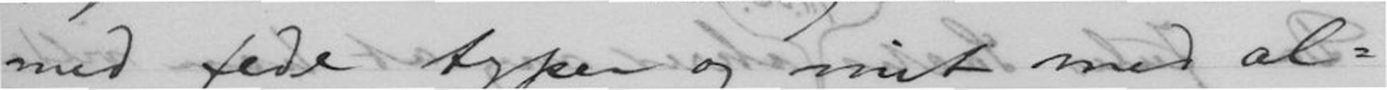med fede typer og mit med al-
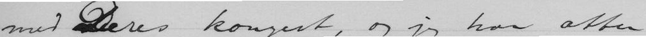med Deres konzert, og jeg har atter
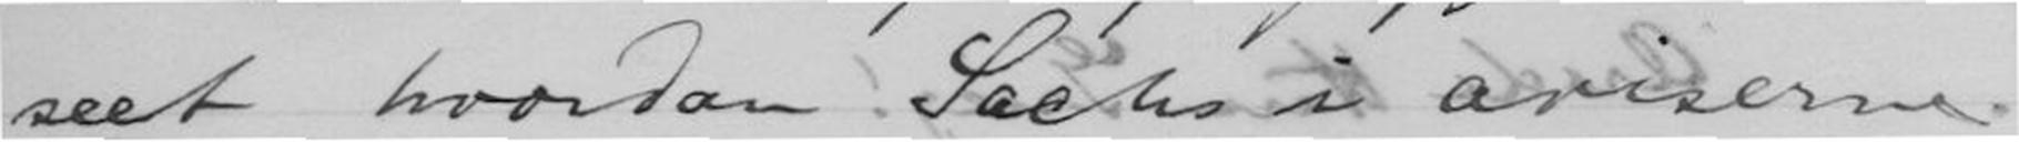seet hvordan Sachs i aviserne
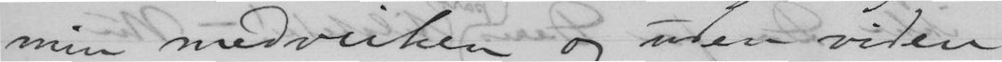min medvirken og uden viden
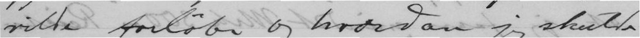vilde forløbe og hvordan jeg skulde
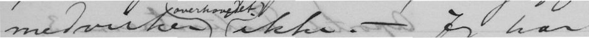medvirker ikke. - Jeg har
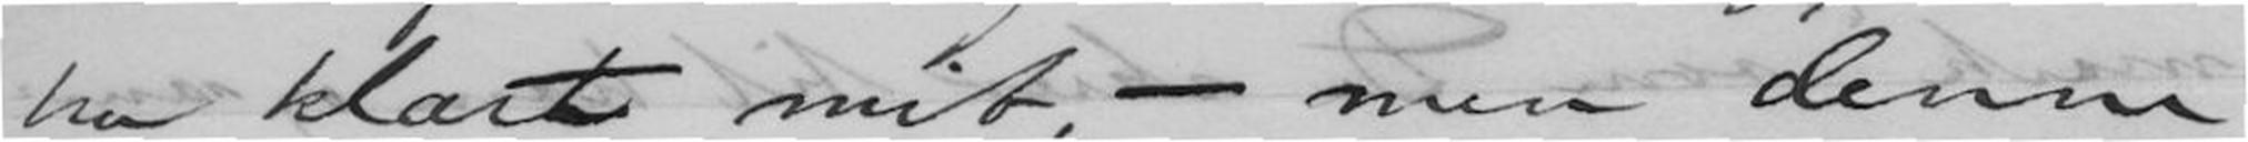ha klart mit , - men denne
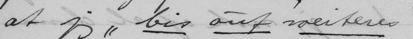at jeg "bis auf weiteres
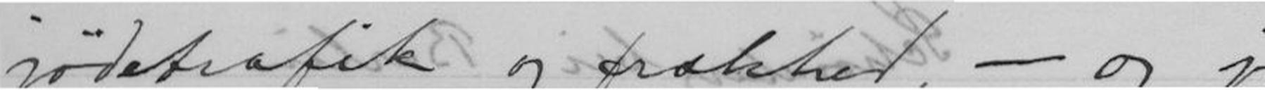jødetrafik og frækhed, - og jeg
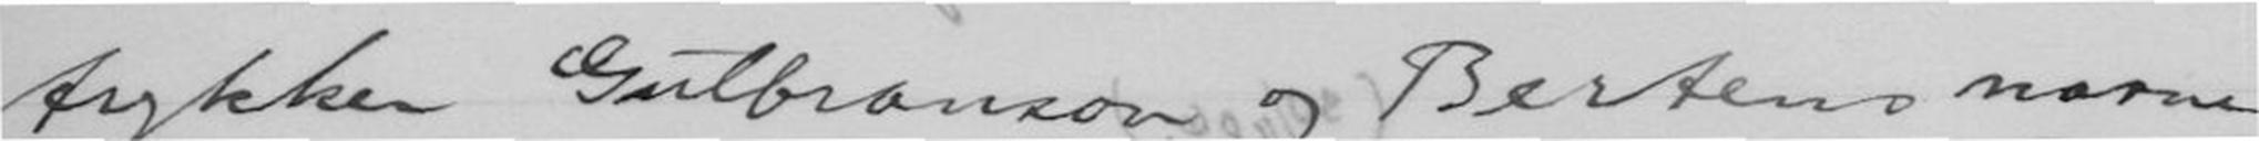trykker Gulbranson og Bertens navne
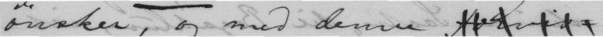og ønsker, og med denne
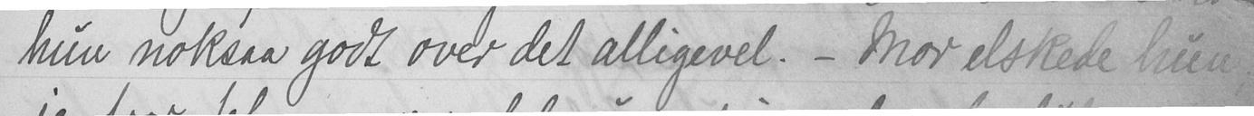hun noksaa godt over det alligevel. - Mor elskede hun,
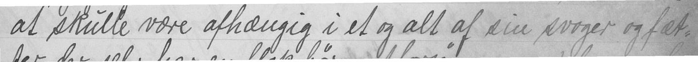at skulle være afhængig i et og alt af sin svoger og fæt-
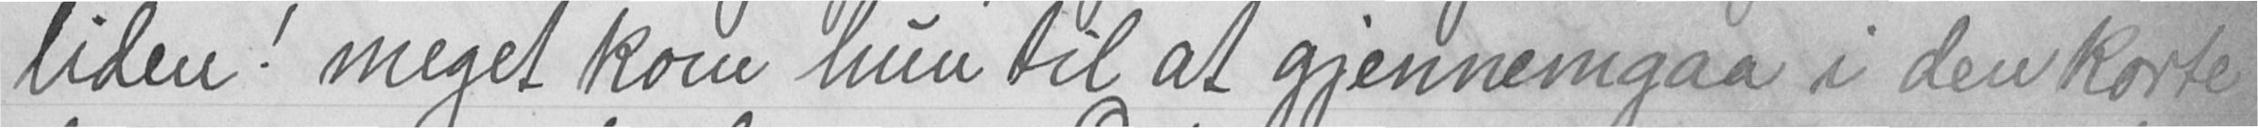liden! meget kom hun til at gjennemgaa i den korte
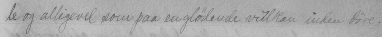le og alligevel som paa en glødende vulkan inden døre.
